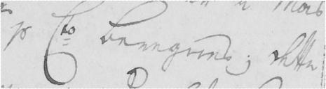p Eto beregnes; dette
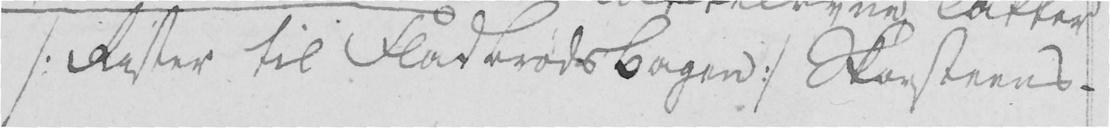/: Rester til Fladbrødsbagen :/ Skorsteens-
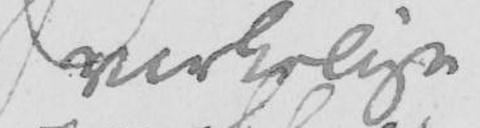virkelige
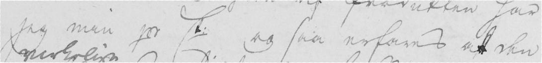jeg min pr E. og saa erfares at den
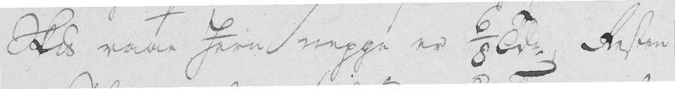Skpd raae Jern neppe er 6/8 Tdr. Resten
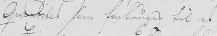Qvantitet som forbruges til et
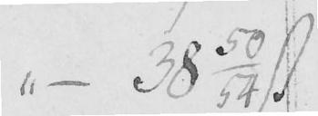"- 38 50/54 s
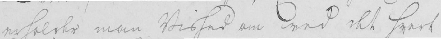erholder man Vished om ved det hvert
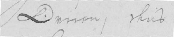Ovnen, dens
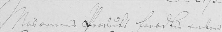Masovnens Produkt forædles enten
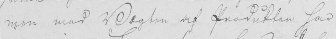men med Vægten af Produkten har
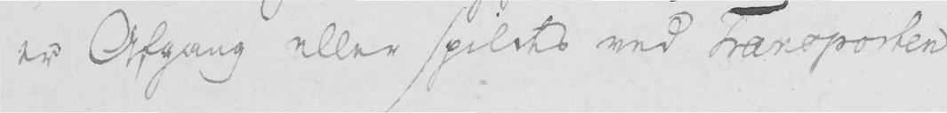er Afgang eller spildes ved Tansporten
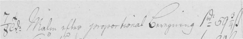7/8 Tdr Malm efter proportional Beregning 1 rde 59 3/4 s
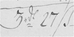3 rde 27 s
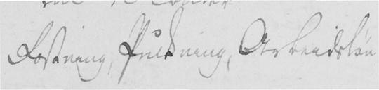Fæstning, Prudning, Arbeidsløn
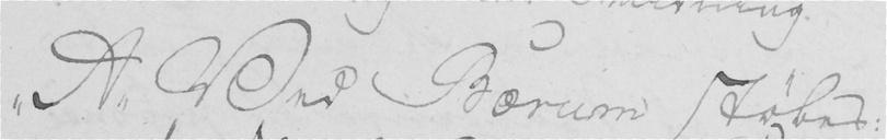"A" Ved Bærum støbes:
